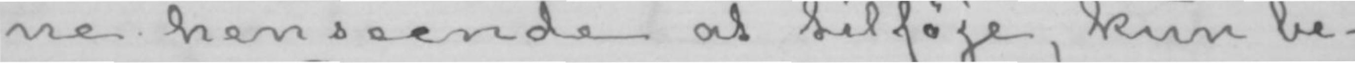ne henseende at tilføje, kun be-
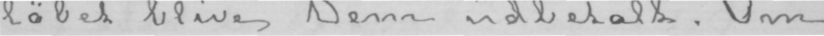løbet blive Dem udbetalt. Om
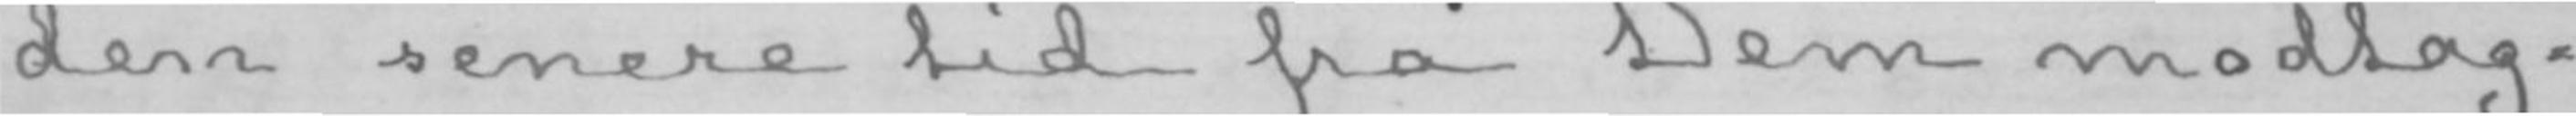den senere tid fra Dem modtag-
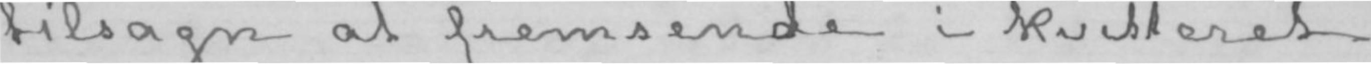tilsagn at fremsende i kvitteret
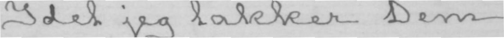Idet jeg takker Dem
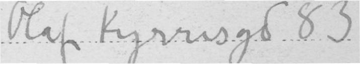Olaf Kyrresgd 83
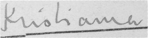Kristiania
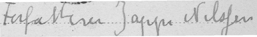Forfatteren Jappe Nilssen
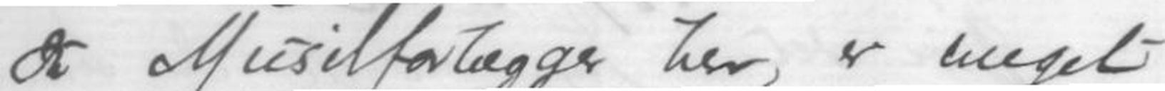Musikforlægger her, er meget
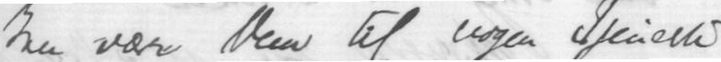kan være Dem til nogen Tjeneste
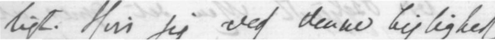ligt. Hvis jeg ved denne lejlighed
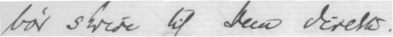bør skrive til dem direkte.
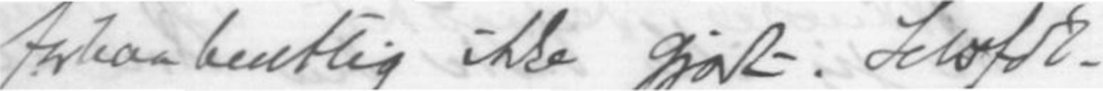forhaabentlig ikke gjort. Selvføl-
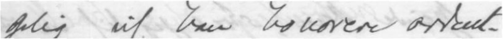gelig vil han ha ordent-
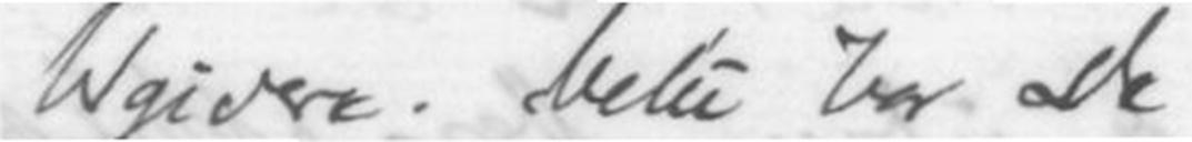Utgivere. Dette har de
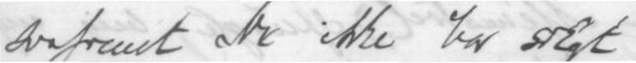saafremt ikke har sligt
